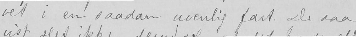vet i en saadan uvenlig fart. De saa
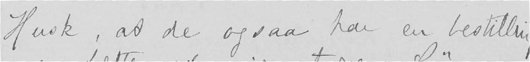Husk, at de ogsaa har en bestilling
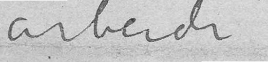arbeide –
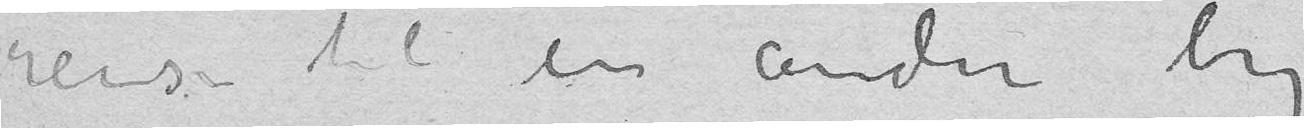reise til en anden by
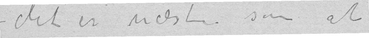– det er næsten som at
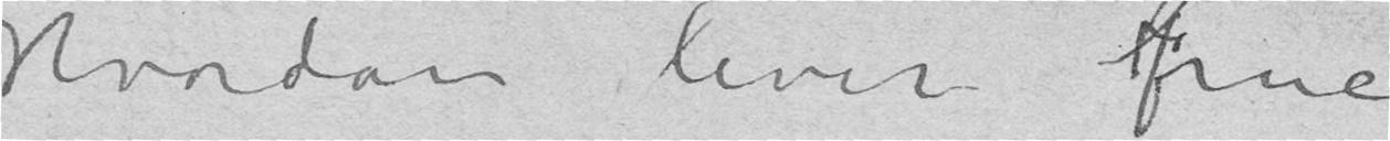Hvordan lever fruen
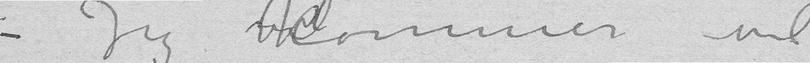– Jeg kommer vel
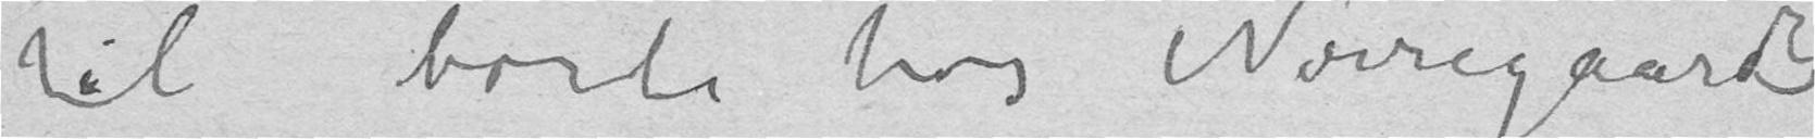til borte hos Nørregaards
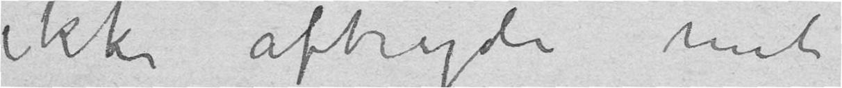ikke afbryde mit
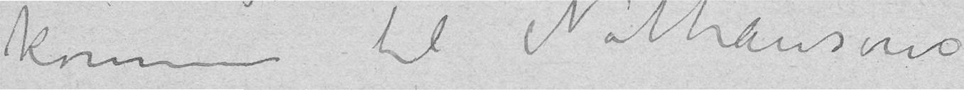komme til Nathansens
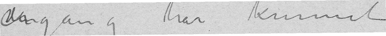engang har kunnet
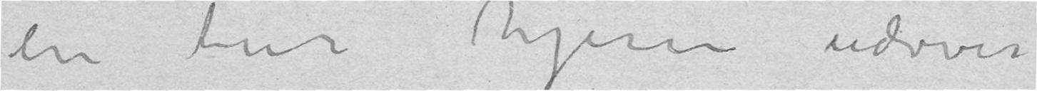en tur hjem udover
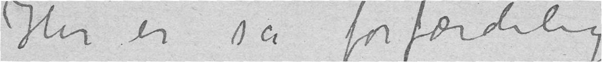Her er så forfærdelige
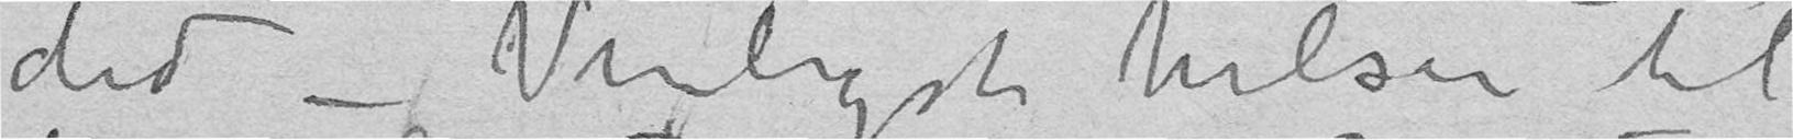did –Venligst hilsen til
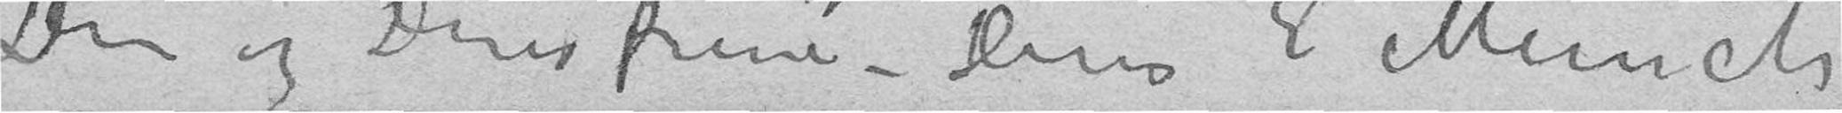Dem og Deres Frue – Deres E Munch
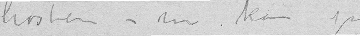høsten – nu kan jeg
